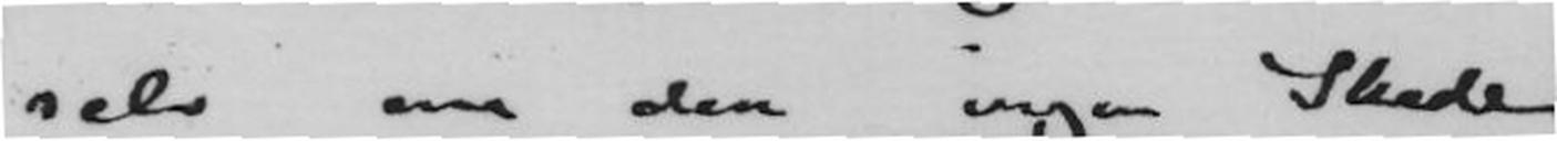selv om den ingen Skade
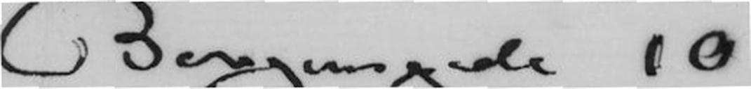Bergensgade 10
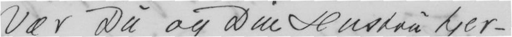Vær Du og Din Hustru hjer-
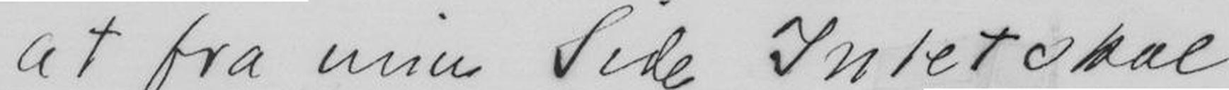at fra min Side Intet skal
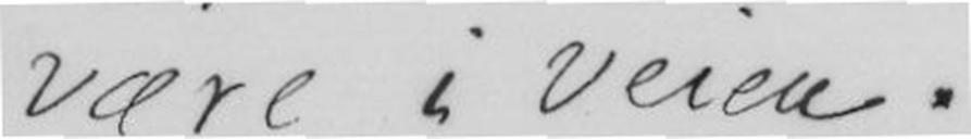være i veien.
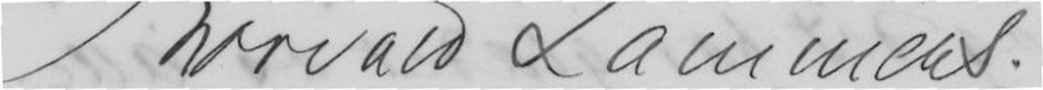Thorvald Lammers
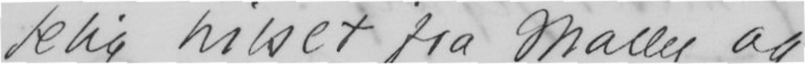telig Hilset fra Mally og
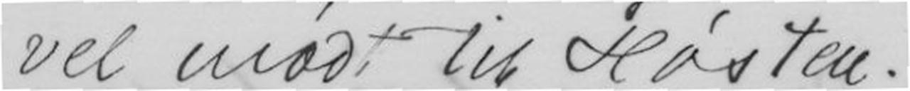vel mødt til Høsten.
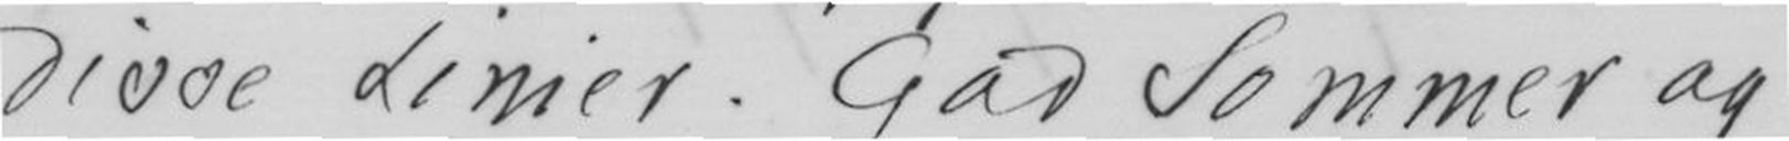disse Linier. God Sommer og
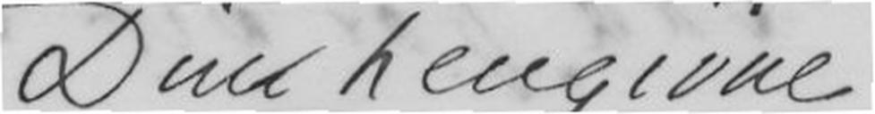Din hengivne
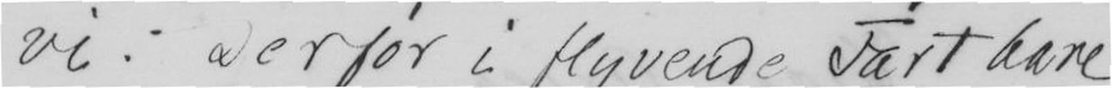vi, Derfor i flyvende Fart bare
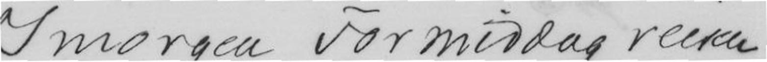Imorgen Formidag reiser
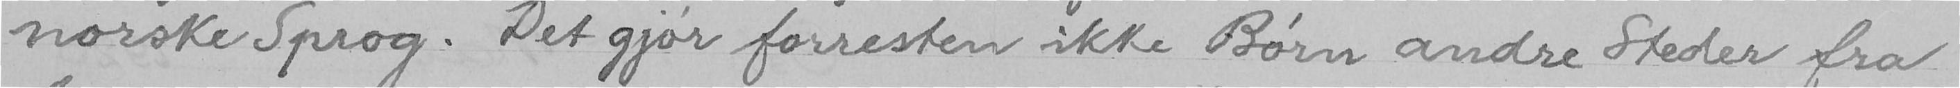norske Sprog. Det gjør forresten ikke Børn andre Steder fra
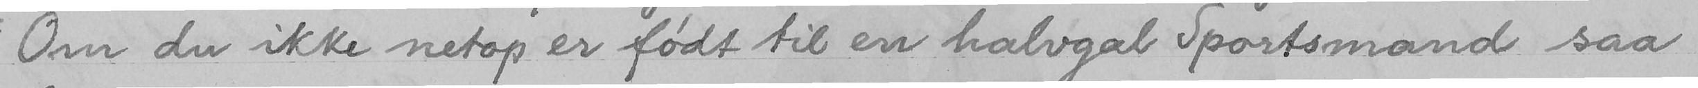Om du ikke netop er født til en halvgal Sportsmand saa
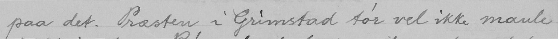paa det. Præsten i Grimstad tør vel ikke maule
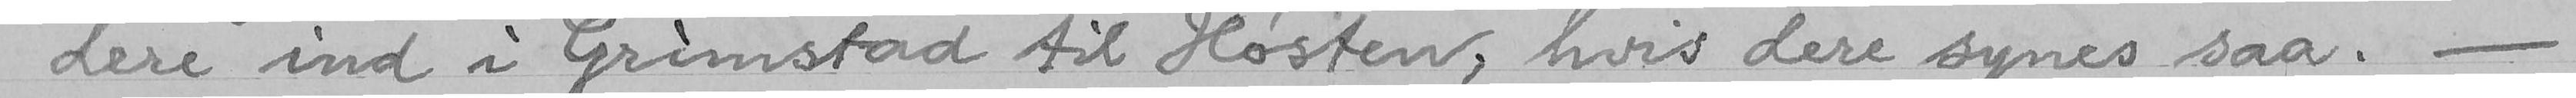dere ind i Grimstad til Høsten, hvis dere synes saa. -
